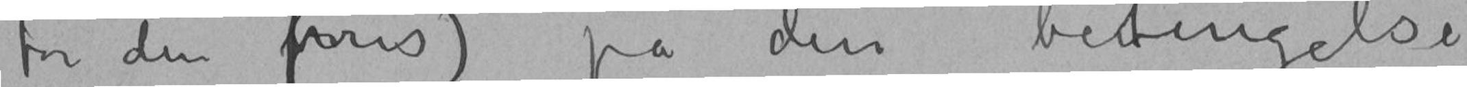for den pris) på den betingelse
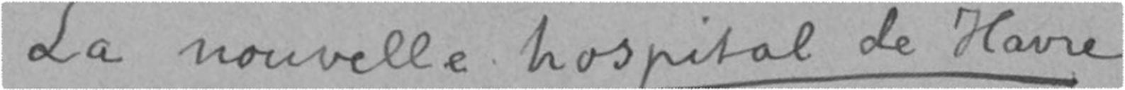La nouvelle hospital de Havre
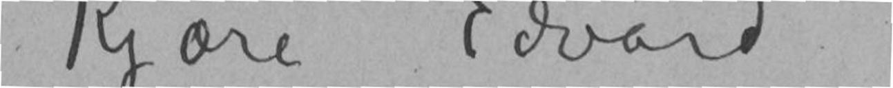Kjære Edvard
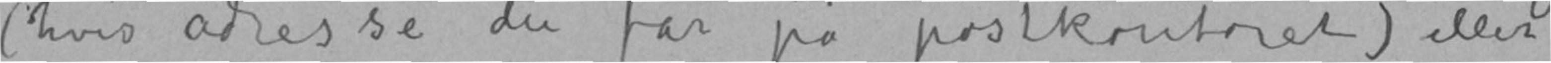(hvis adresse du får på postkontoret) eller
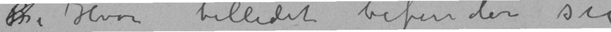Bi Hvor billedet befinder sig
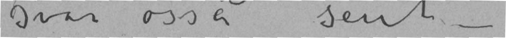svar osså sent –
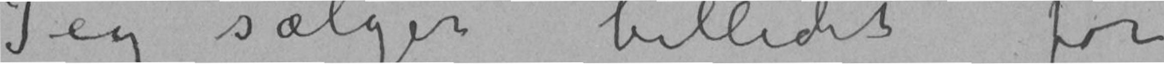Jeg sælger billedet for
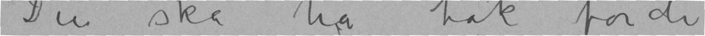Du ska ha tak fordi
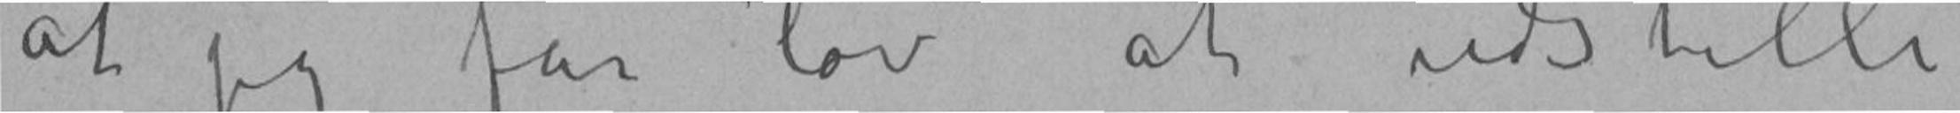at jeg får lov at udstille
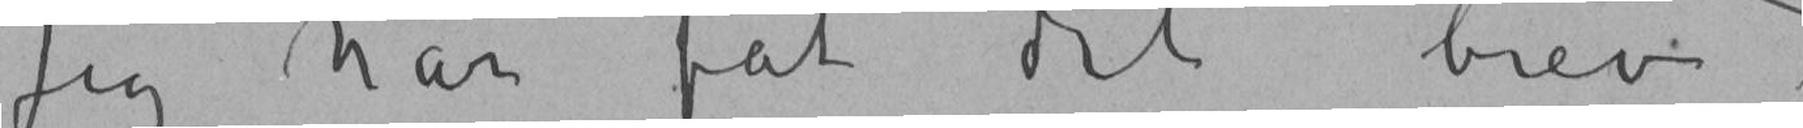Jeg har fåt dit brev
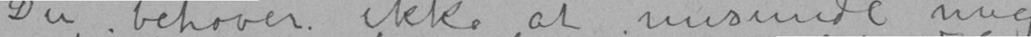Du behøver ikke at misunde mig
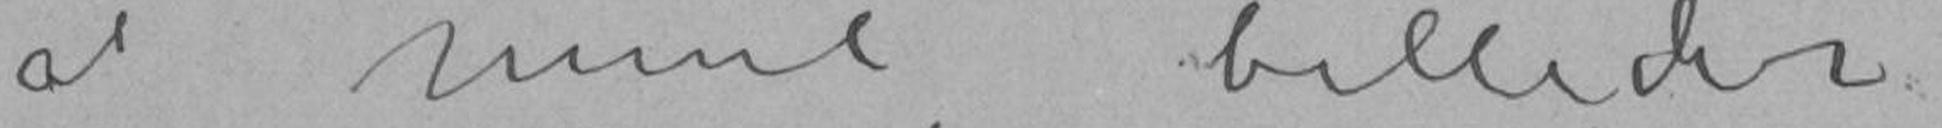af mine billeder
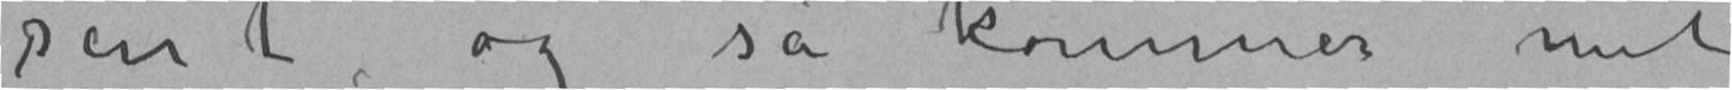sent og så kommer mit
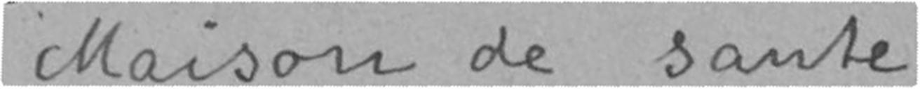Maison de sante
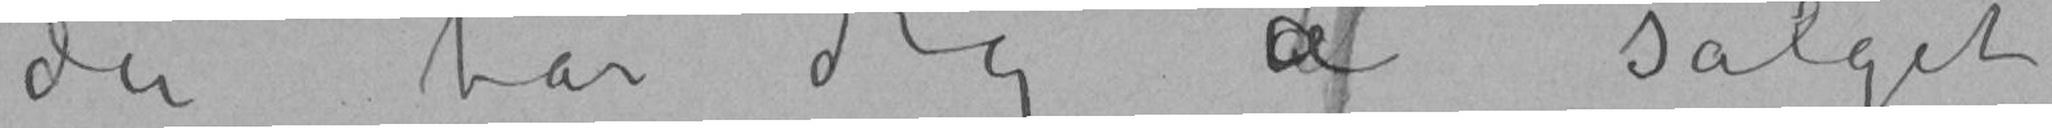du tar dig af salget
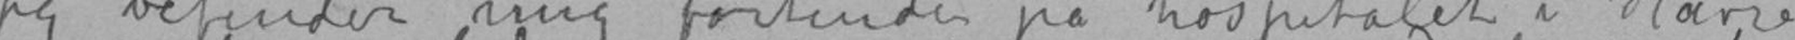jeg befinder mig fortinden på hospitalet i Havre
